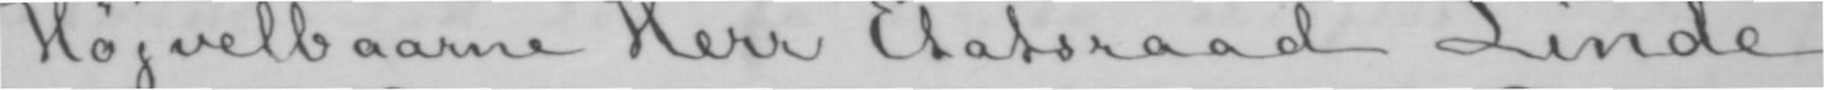Højvelbaarne Herr Etatsraad Linde,
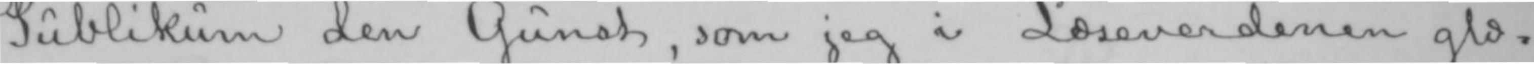Publikum den Gunst, som jeg i Læseverdenen glæ-
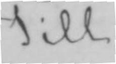Till
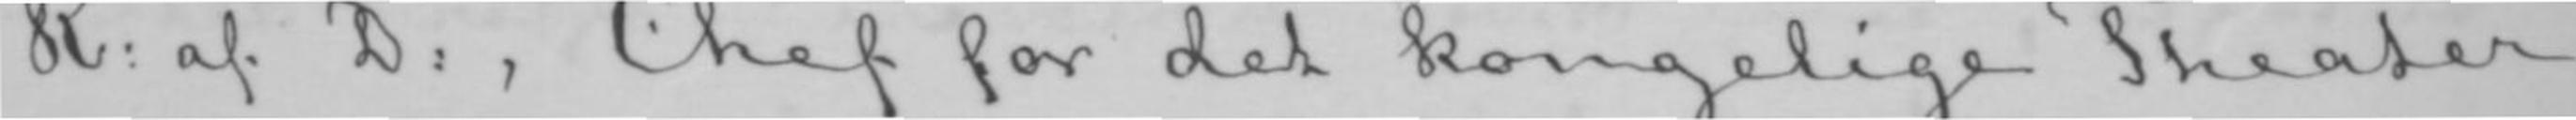R: af D:, Chef for det kongelige Theater
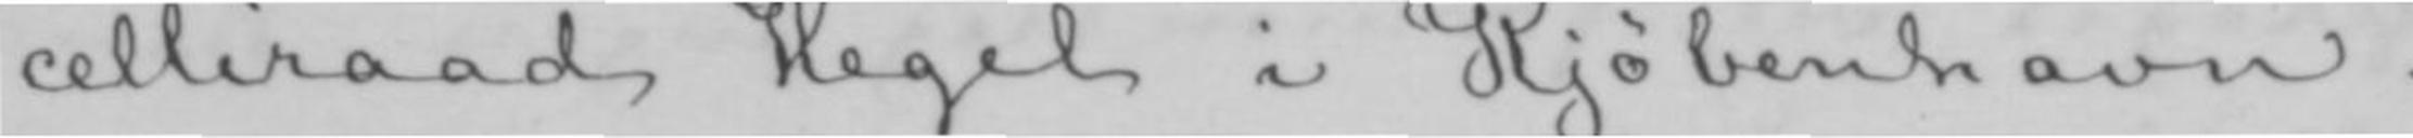celliraad Hegel i Kjøbenhavn.
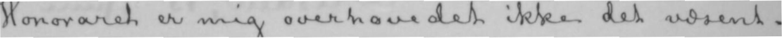Honoraret er mig overhovedet ikke det væsent-
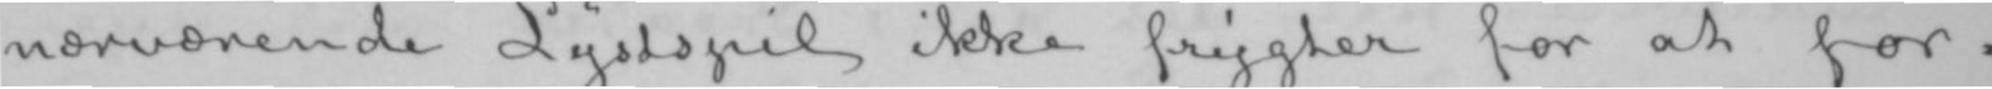nærværende Lystspil ikke frygter for at for-
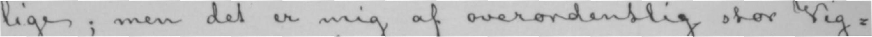lige; men det er mig af overordentlig stor Vig-
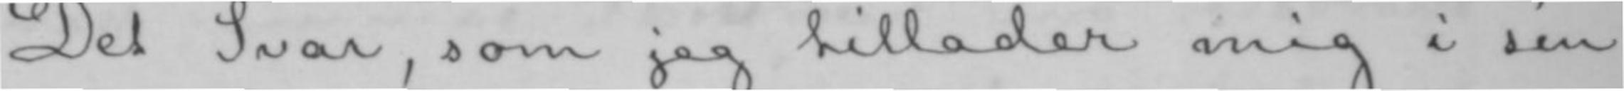Det Svar, som jeg tillader mig i sin
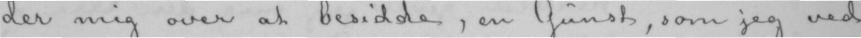der mig over at besidde, en Gunst, som jeg ved
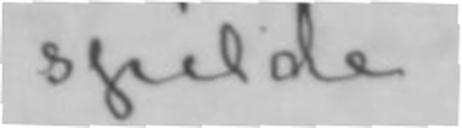spilde.
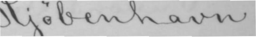Kjøbenhavn.
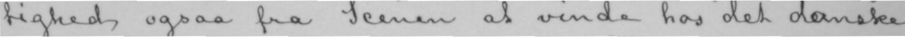tighed ogsaa fra Scenen at vinde hos det danske
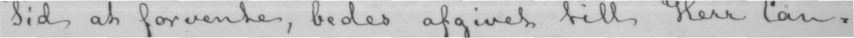Tid at forvente, bedes afgivet till Herr Can-
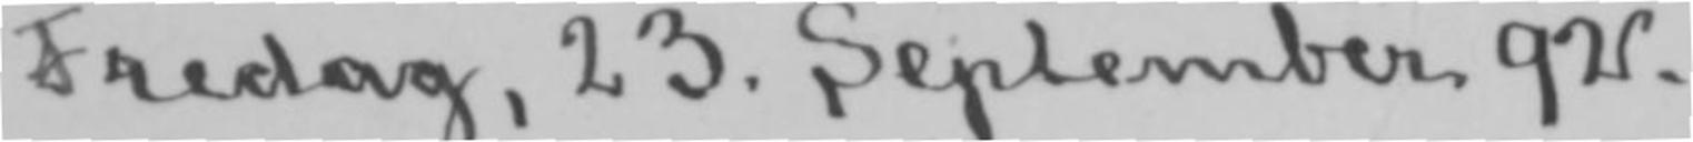Fredag, 23. September 92.
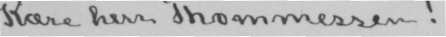Kære herr Thommessen!
